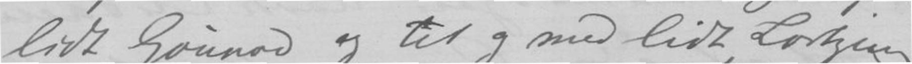lidt Gounod og til og med lidt Lortzing!
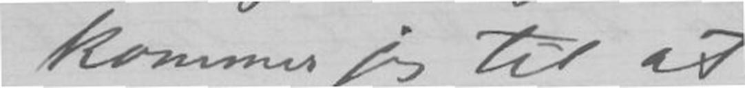kommer jeg til at
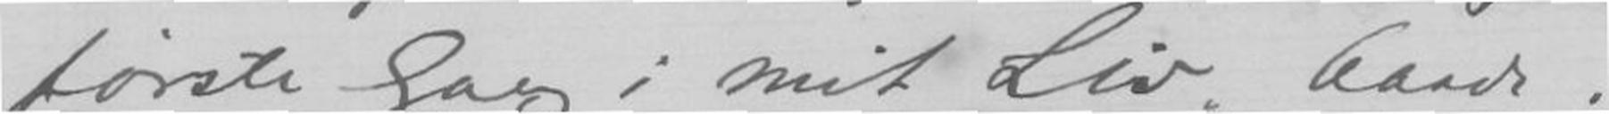første Gang i mit Liv baade i
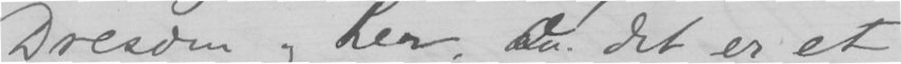Dresden og her. O! det er et
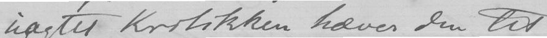uagtet Kritikken hæver den til
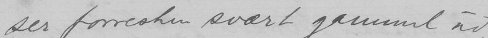ser forresten svært gammel ud,
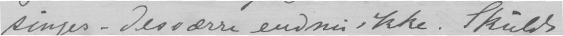singes- desværre endnu ikke. Skulde
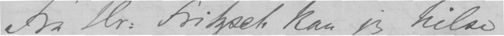Fra Hr. Fritzsct kan jeg hilse
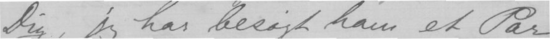Dig, jeg har besøgt ham et Par
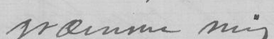græmme mig.
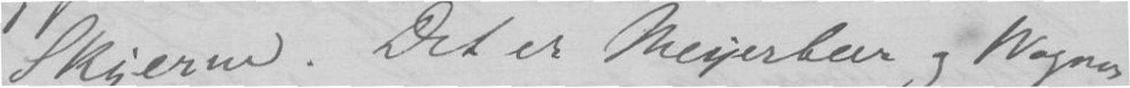Skjerne. Det er Meyerbeer og Wagner
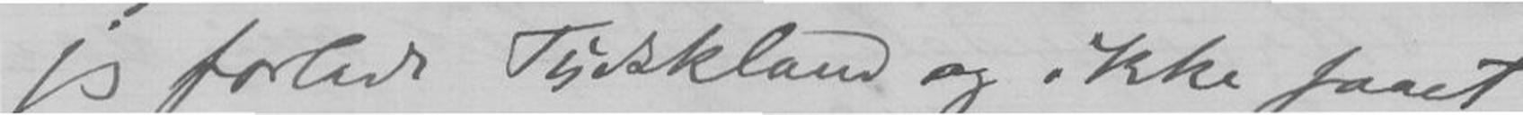jeg forlade Tydskland og ikke faaet
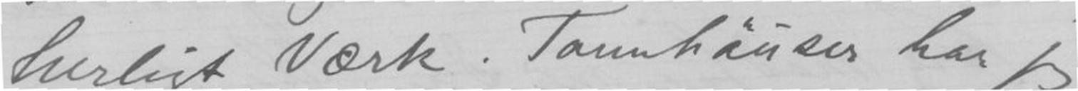herligt Værk. Tannhäuser har jeg
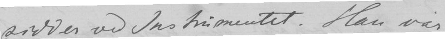sidder ved Instrumentet. Han var
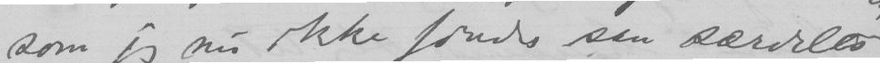som jeg nu ikke foruds saa særdeles
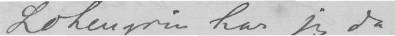Lohengrim har jeg da
